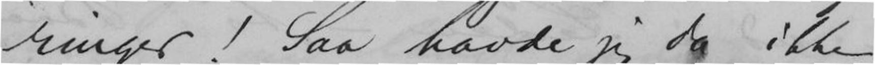ringer! Saa havde jeg da ikke
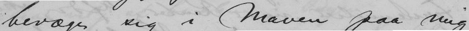bevæge sig i maven paa mig
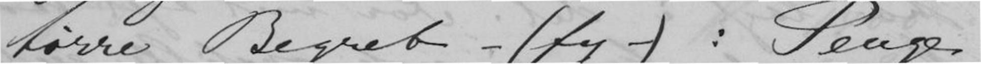tørre Begreb - (fy -): Penge.
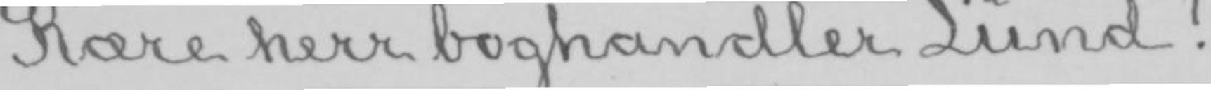Kære herr boghandler Lund!
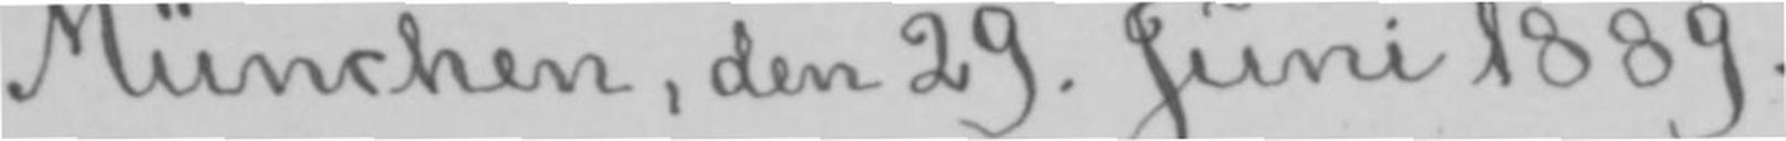München, den 29. Juni 1889.
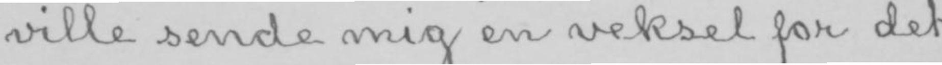ville sende mig en veksel for det
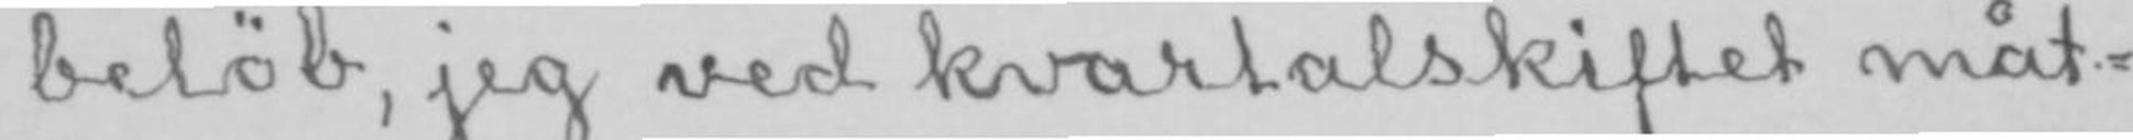beløb, jeg ved kvartalskiftet måt-
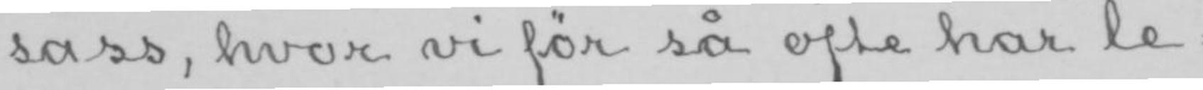sass, hvor vi før så ofte har le-
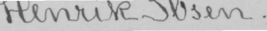Henrik Ibsen.
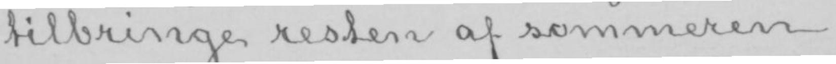tilbringe resten af sommeren
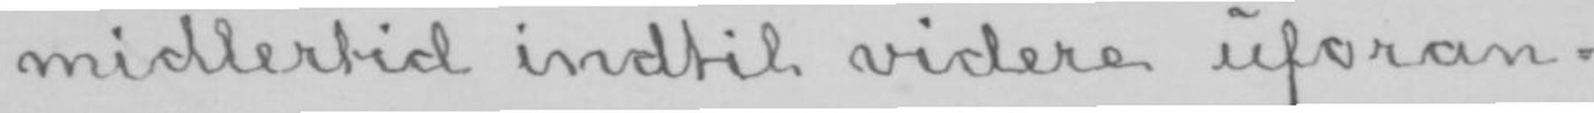midlertid indtil videre uforan-
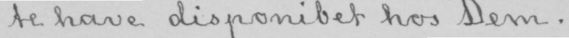te have disponibet hos Dem.
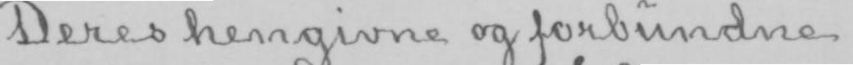Deres hengivne og forbundne
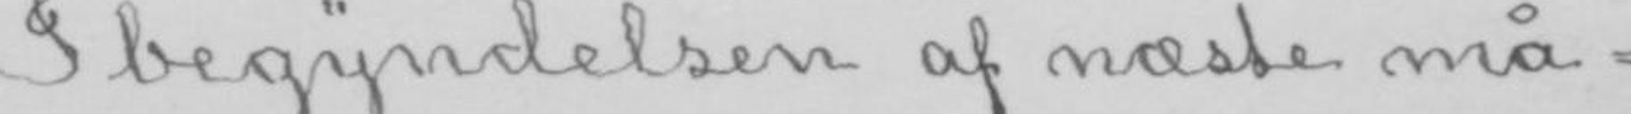I begyndelsen af næste må-
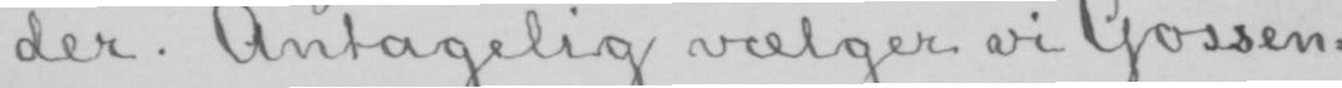der. Antagelig vælger vi Gossen-
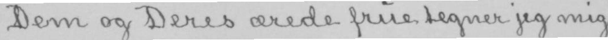Dem og Deres ærede frue tegner jeg mig
